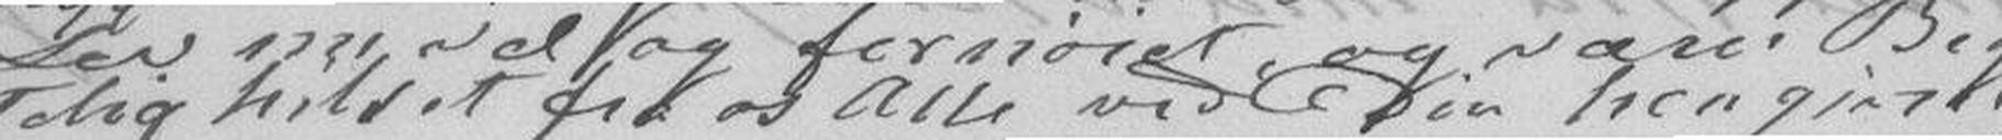telig hilset fra os Alle ved Din hengivne
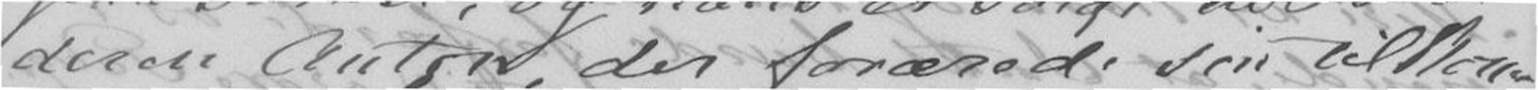deren Anton, der forærede sin til kom-
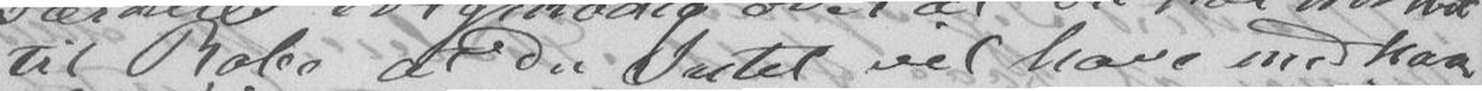til Rabe at du Intet vil have med han
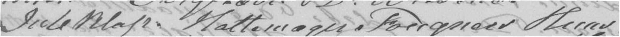Juleklap. Hattemager Fougners Huus
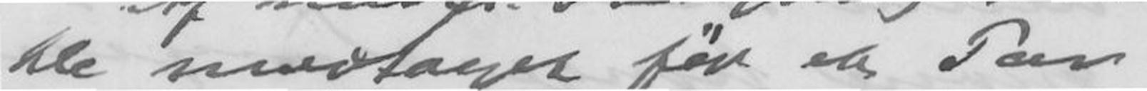De modtaget før et Par
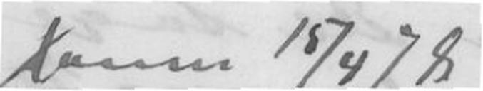Xania 15/4 78
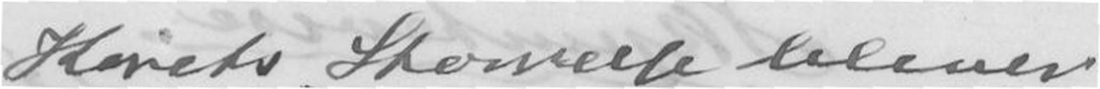Korets Størrelse bliver
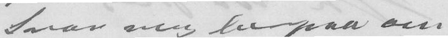Svar mig herpaa om
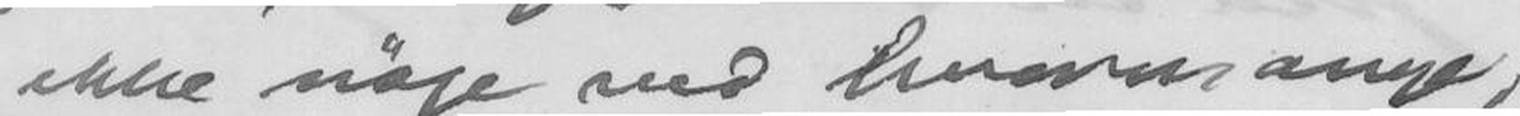ikke nøje ved hvormange,
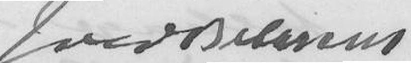Joh D Behrens
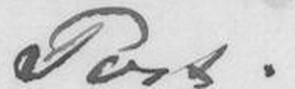Post.
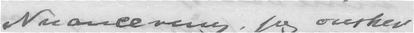Nuancering jeg ønsker
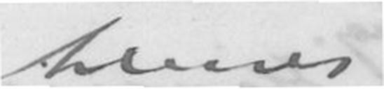Deres
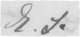E. S.
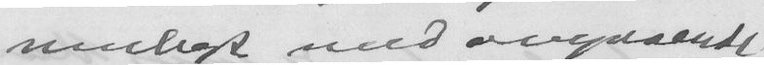muligt med omgaaende
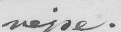rejse.
